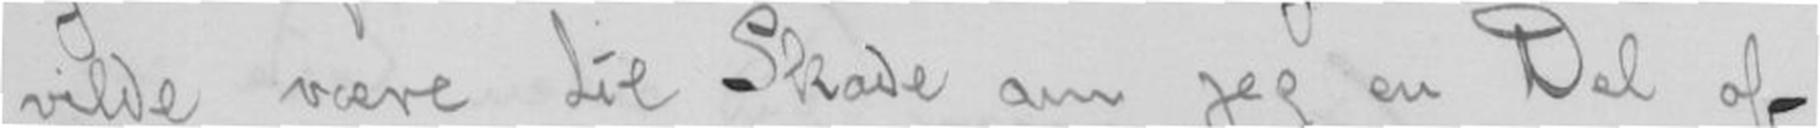ikke vilde være til Skade om jeg en Del af
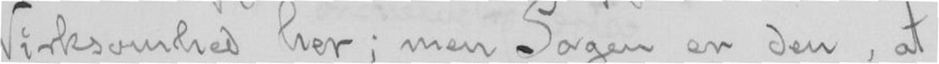Virksomhed her; men Sagen er den, at
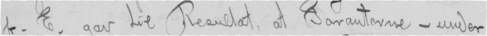f. E. gav til Resultat, at Garanterne - under
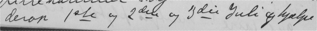derop 1ste og 2den og 3die Juli og hjælpe
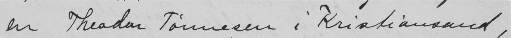en Theodor Tønnesen i Kristiansand,
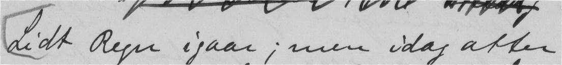Lidt Regn igaar; men idag atter
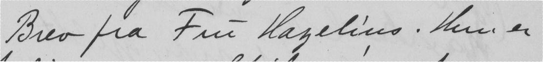Brev fra Fru Hagelius. Hun er
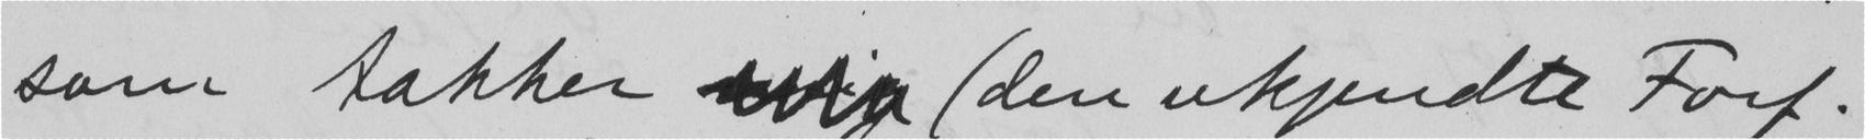som takker  den ukjendte Forf.
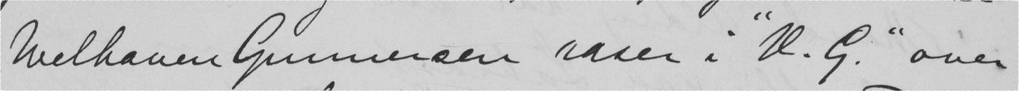Welhaven Gunnersen raser i "V. G." over
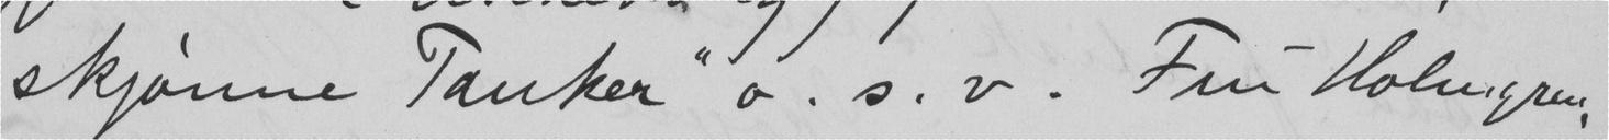skjønne Tanker" o. s. v. Fiu Holmgren
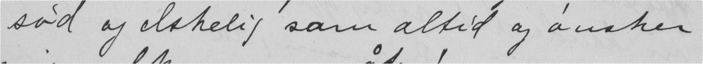sød og eskelig som altid og ønsker
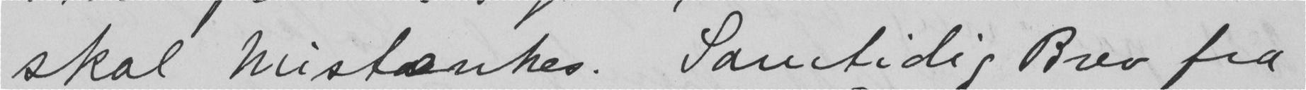skal mistænkes. Samtidig Brev fra
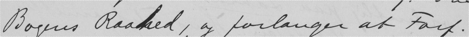Bogens Raahed, og forlanger at Forf.
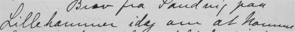Lillehammer idag om at komme
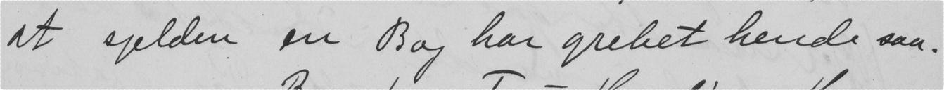at sjelden en Bog har grebet hende saa.
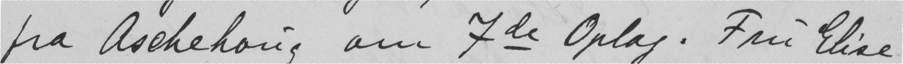fra Aschehoug om 7de Oplag. Fru Elise
